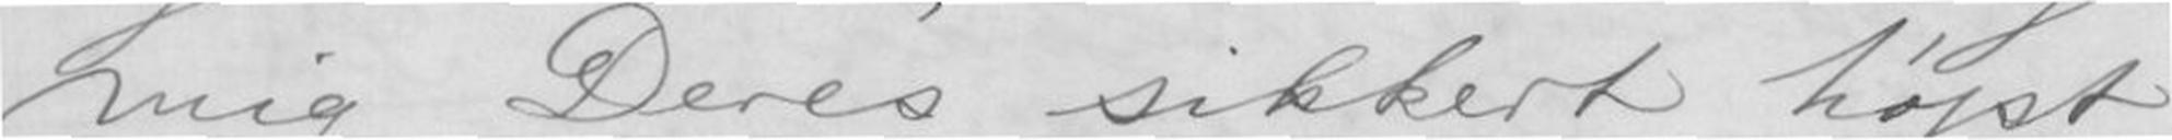mig Deres sikkert høyst
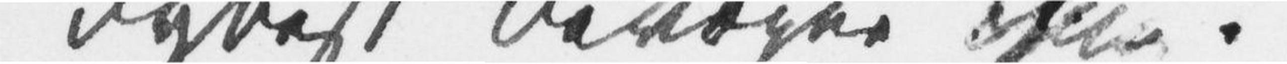dybest bevæger En.
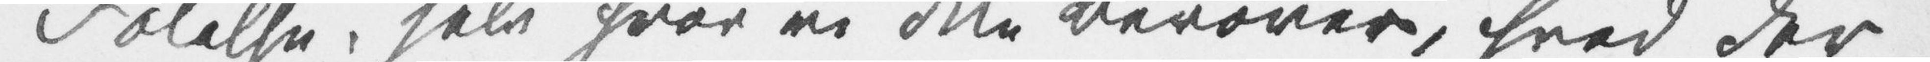Følelse, selv hvor vi ikke berører, hvad der
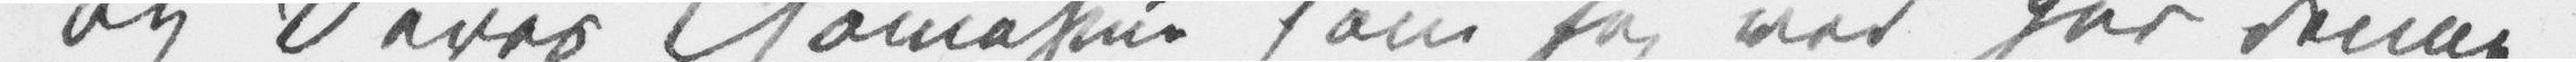og Deres Thomasine som jeg ved har denne
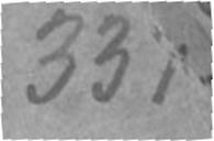337.
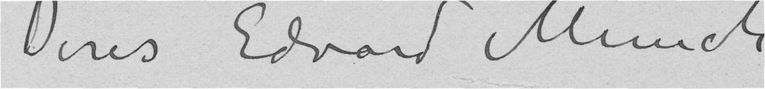Deres Edvard Munch
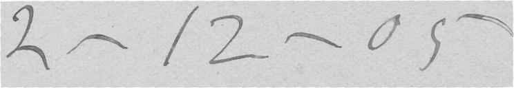2-12-05
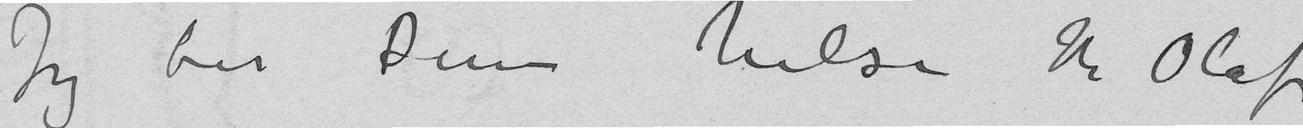Jeg ber Dem hilse Hr Olaf
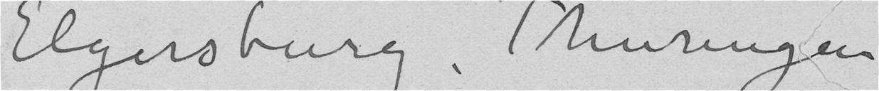Elgersburg, Thüringen
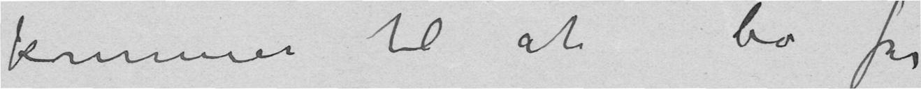kommer til at bo får
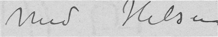Med Hilsen
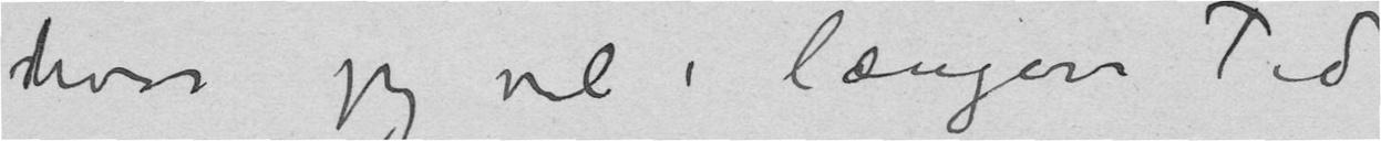hvor jeg vel i længere Tid
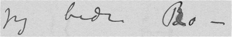jeg bedre Ro –
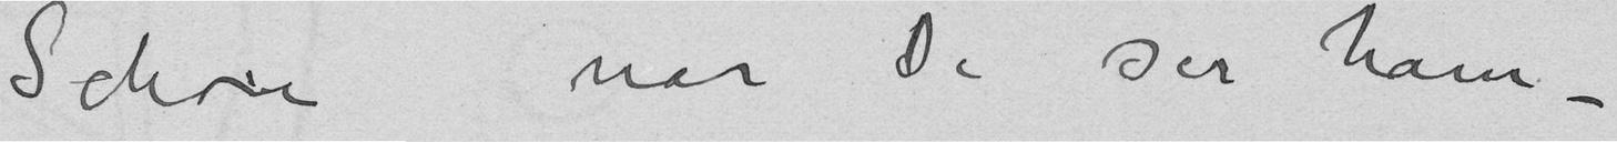Schou når De ser ham –
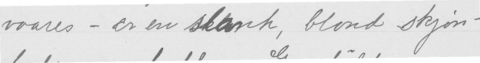vaares - er en slank, blond skjøn-
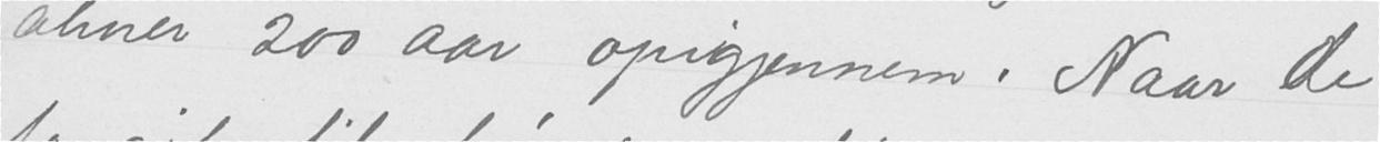ahner 200 aar opigjennem. Naar de
